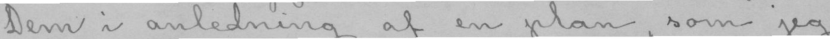Dem i anledning af en plan, som jeg
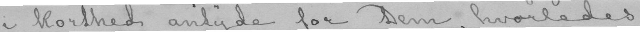i korthed antyde for Dem, hvorledes
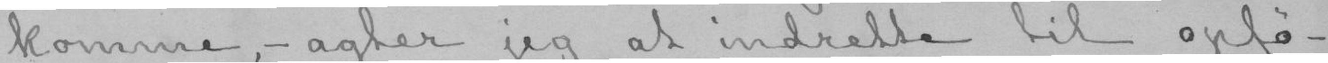komme, - agter jeg at indrette til opfø-
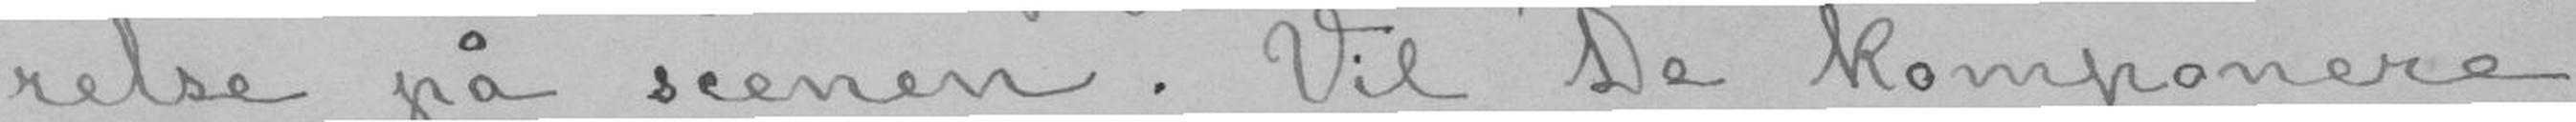relse på scenen. Vil De komponere
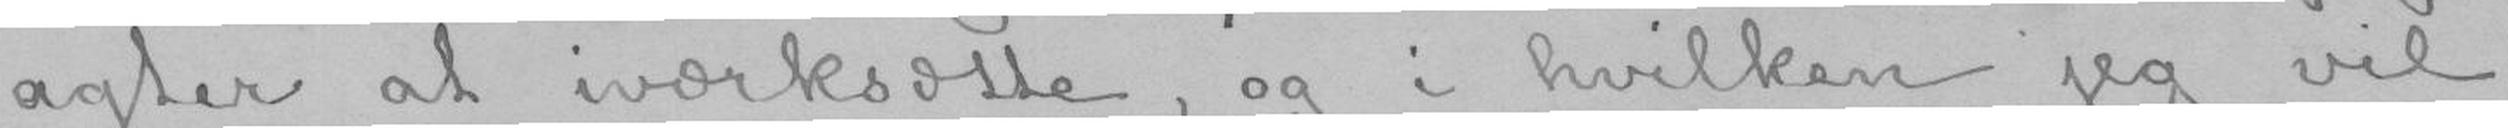agter at iværksætte, og i hvilken jeg vil
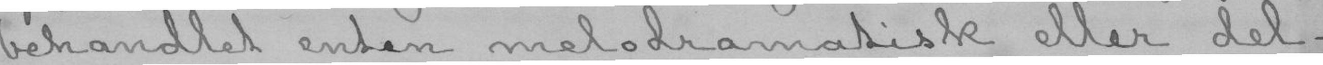behandlet enten melodramatisk eller del-
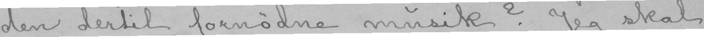den dertil fornødne musik? Jeg skal
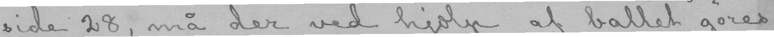side 28, må der ved hjælp af ballet gøres
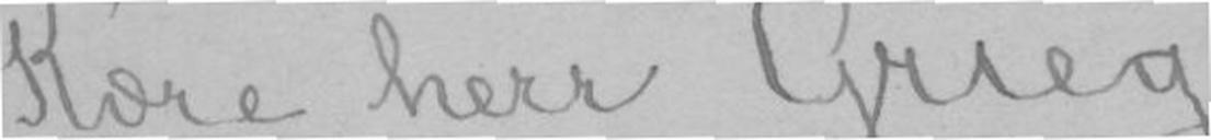Kære herr Grieg
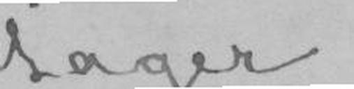tager.
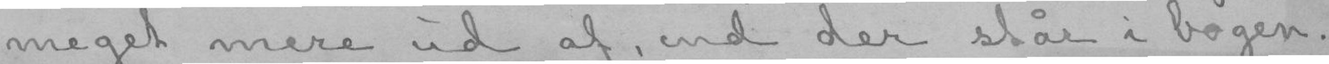meget mere ud af, end der står i bogen.
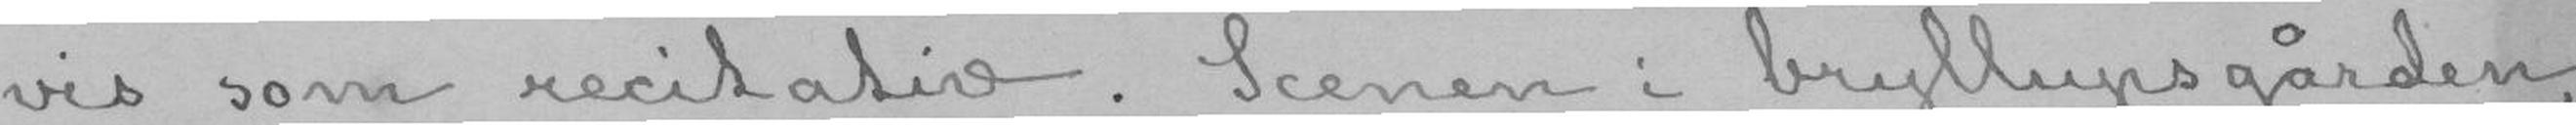vis som recitativ. Scenen i bryllupsgården,
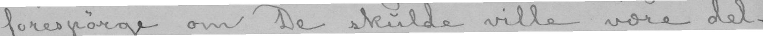forespørge om De skulde ville være del-
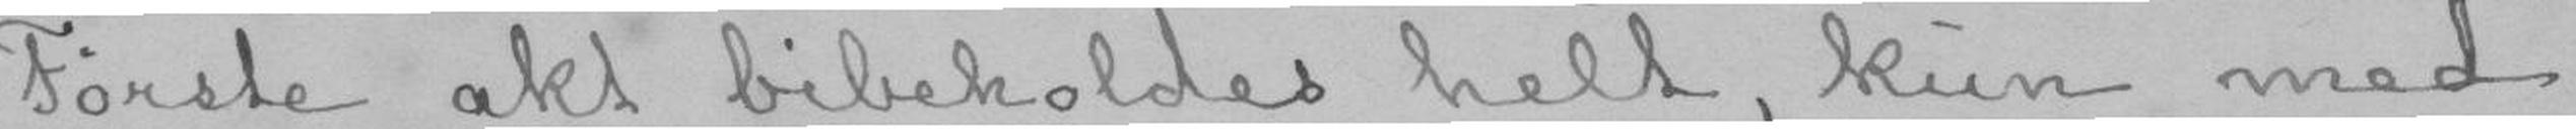Første akt bibeholdes helt, kun med
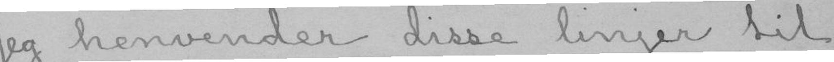Jeg henvender disse linjer til
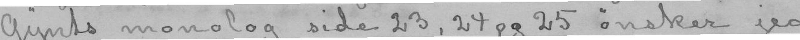Gynts monolog side 23, 24 og 25 ønsker jeg
